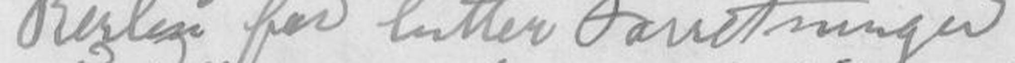Berlin for lutter Forretninger
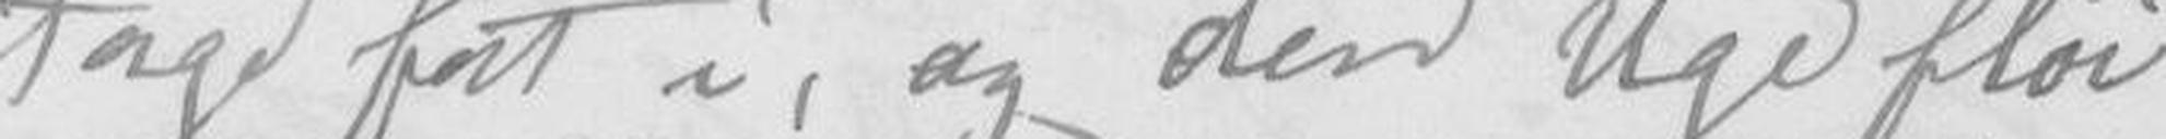tage fat i, og den Uge fløi
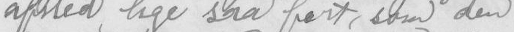afsted lige saa fort, som den
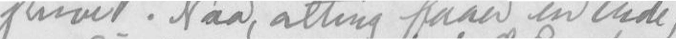skrive. Naa, alting faaer en Ende,
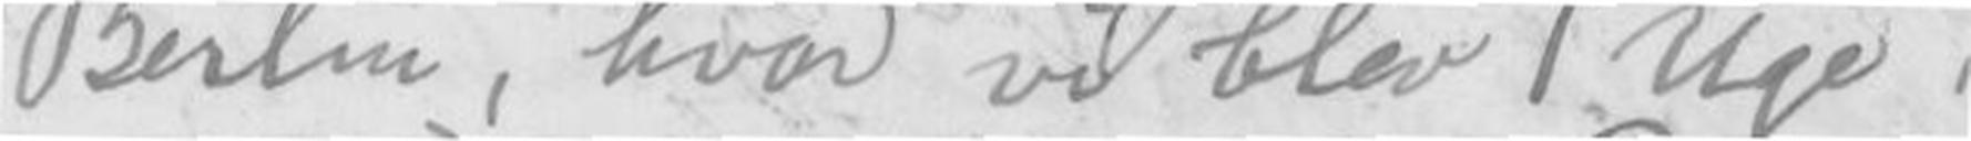Berlin, hvor vi blev 1 Uge,
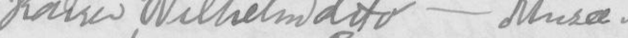Kaiser Wilhelm dito - Music-
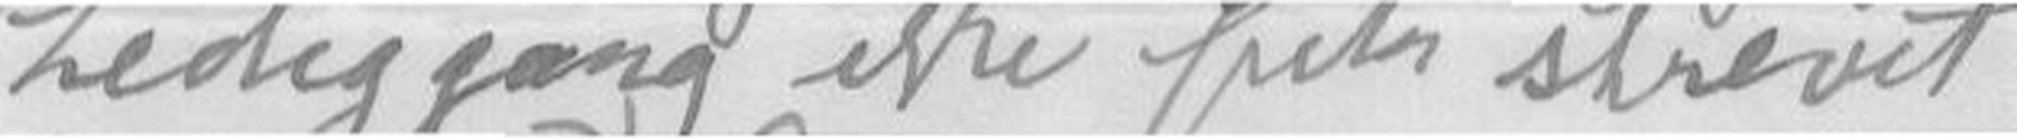Lediggang ikke fik skrevet
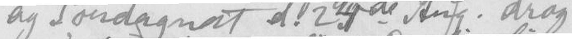og Søndagnat d. 24de Aug. drog
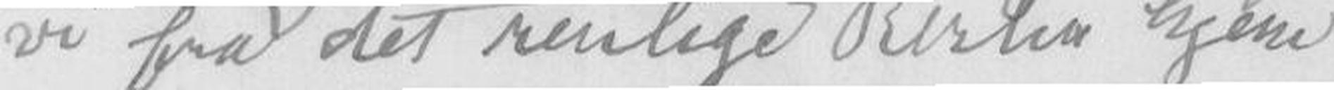vi fra det renlige Berlin hjem
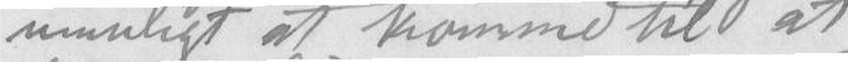umuligt at komme til at
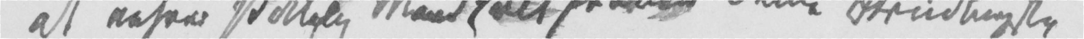at enhver skikkelig Mand vil frabede denne Strindbergske
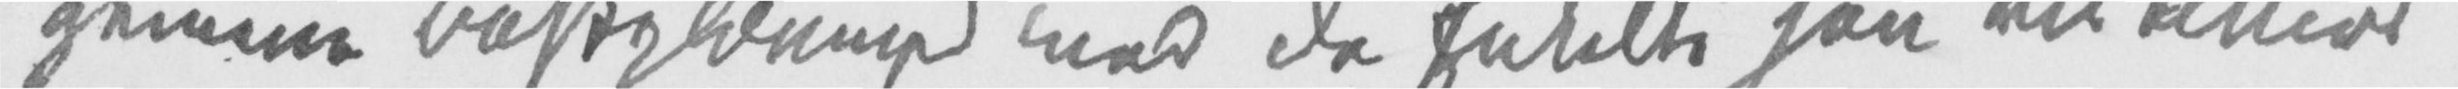gemene Beskyldninger mod de Enkelte han vil tillivs
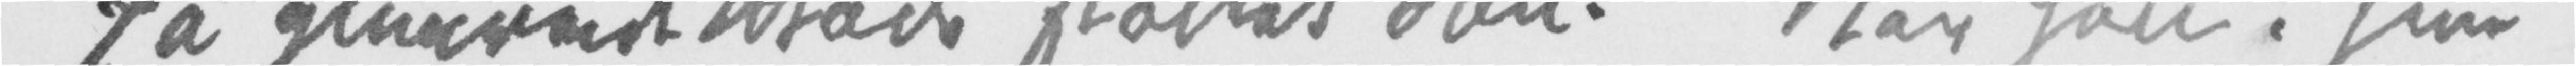på glimrende Måde støttet dem. Når han i sine
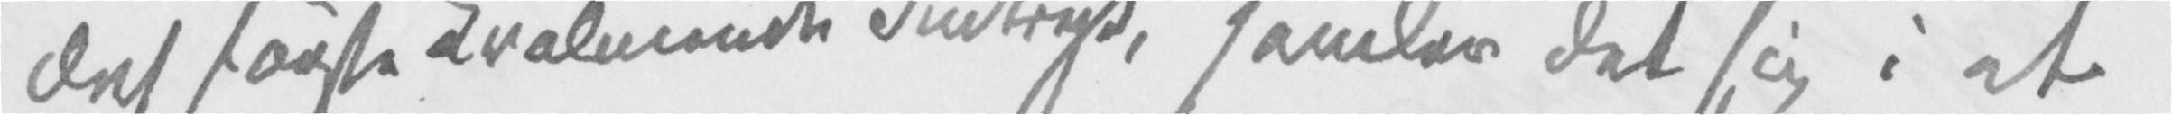det første kvalmende Indtryk, samler det sig i et
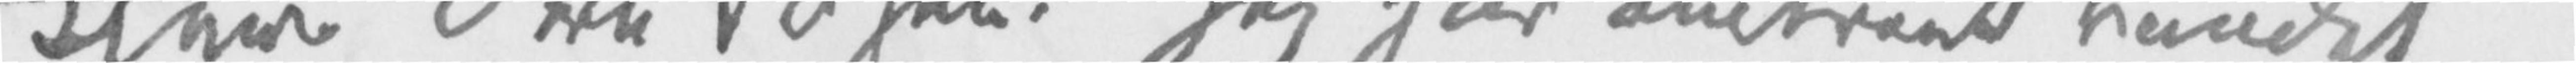Kjere Fru Ibsen. Jeg har omtrent vundet
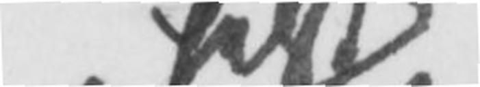helst
